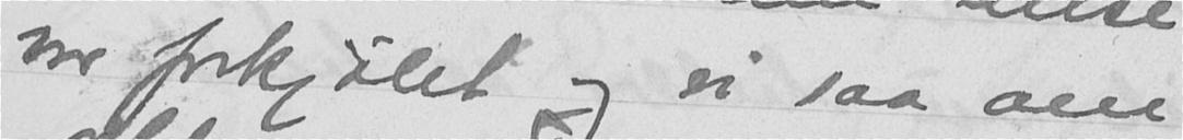var forkjølet og vi saa om
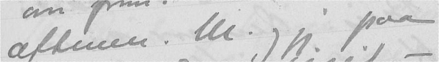aftenen. M. og jeg paa
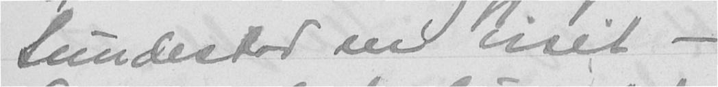Lundestad en visit -
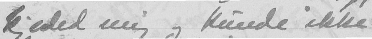kjeded mig og kunde ikke
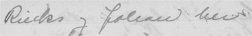Riecks og Johan her
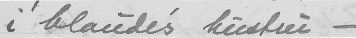i Claude's hustru -
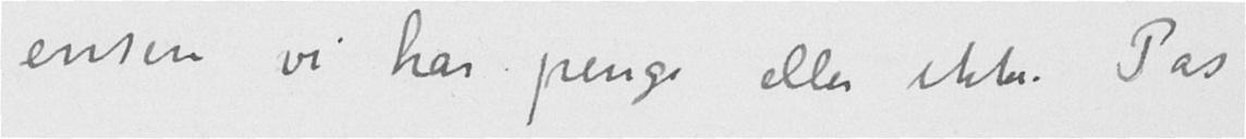enten vi har penge eller ikke. Pas
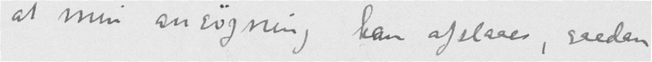at min ansøgning kan afslaaes, saadan
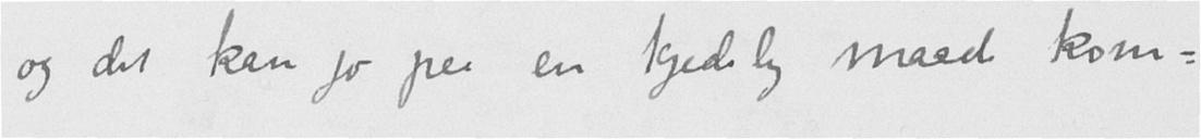og det kan jo paa en kjedelig maade kom-
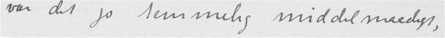var det jo temmelig middelmaadigt,
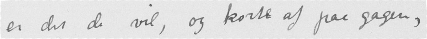er det de vil, og korte af paa gagen,
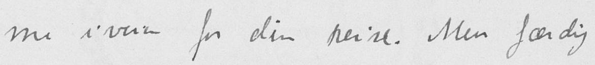me i veien for din reise. Men færdig
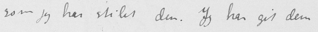som jeg har stilet den. Jeg har git dem
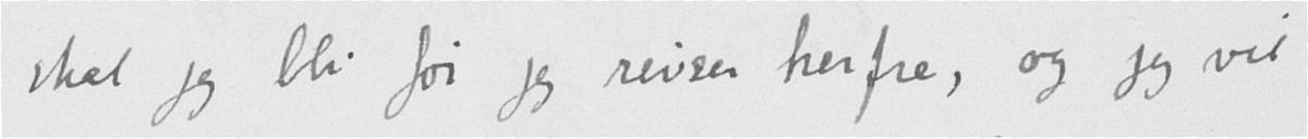skal jeg bli før jeg reiser herfra, og jeg vil
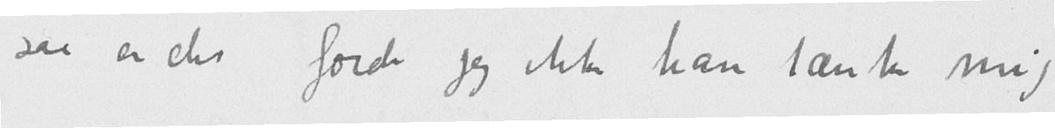saa er det fordi jeg ikke kan tænke mig
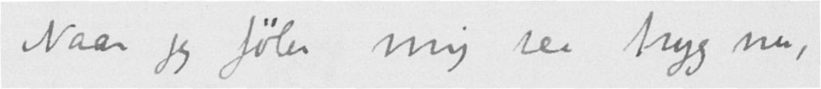Naar jeg føler mig saa tryg nu,
